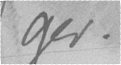ger.
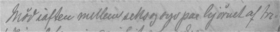Mød iaften mellem seks og syv paa hjørnet af tri-
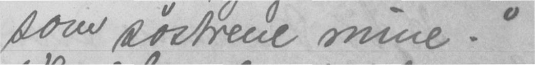som søstrene mine."
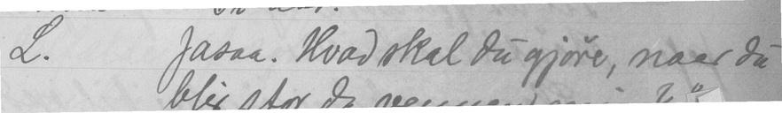L. Jasaa. Hvad skal du gjøre, naar du
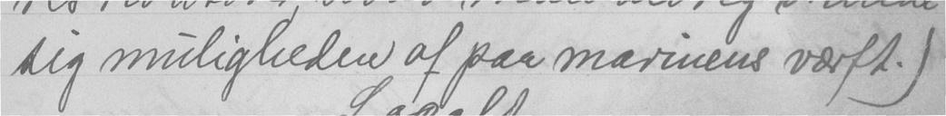sig muligheden af paa marinens værft.)
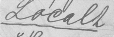Localt
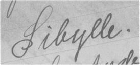Sibylle.
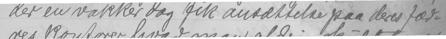der en vakker dag fik ansættelse paa deres fæd-
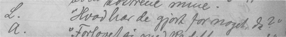L. Hvad har de gjort for noget, da?"
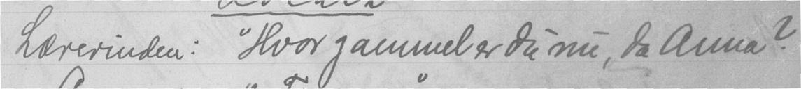Lærerinden: "Hvor gammel er du nu, da Anna?"
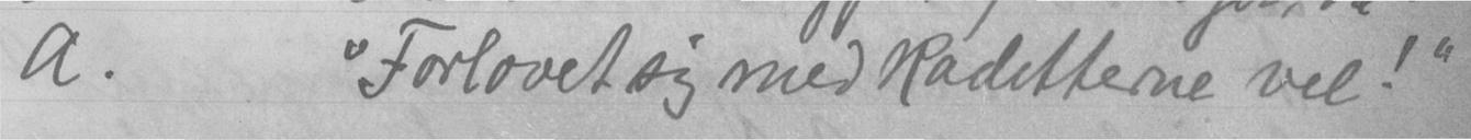A. "Forlovet sig med kadetterne vel!"
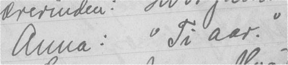Anna: "Ti aar."
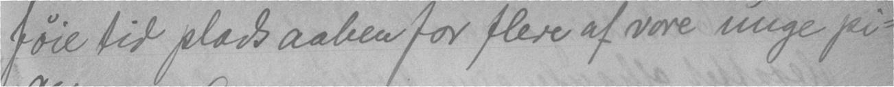føie tid plads aaben for flere af vore unge pi-
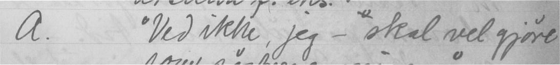A. "Ved ikke, jeg - skal vel gjøre
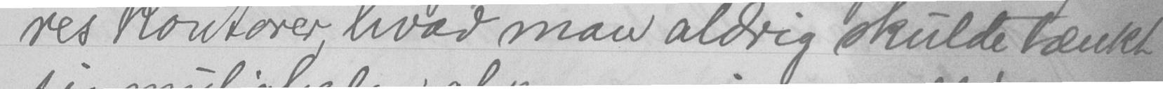res kontorer, hvad man aldrig skulde tænkt
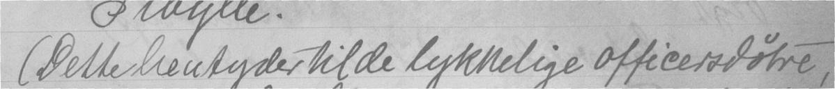(Dette hentyder til de lykkelige officersdøtre,
